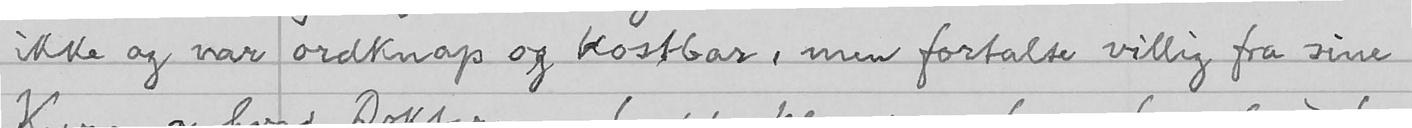ikke og var ordknap og kostbar, men fortalte villig fra sine
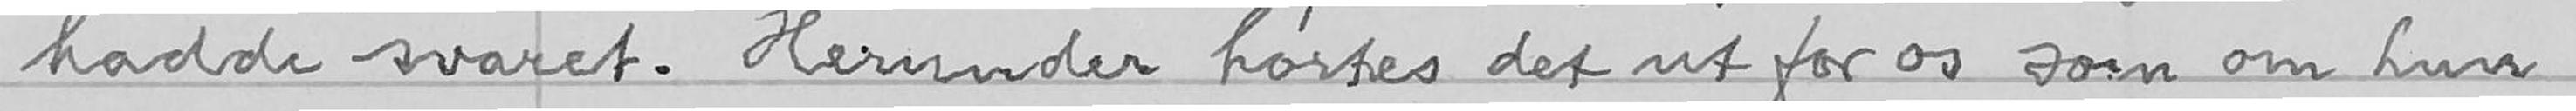hadde svaret. Herunder hørtes det ut for os som om hun
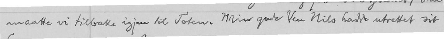maatte vi tilbake igjen til Toten. Min gode Ven Nils hadde utrettet sit
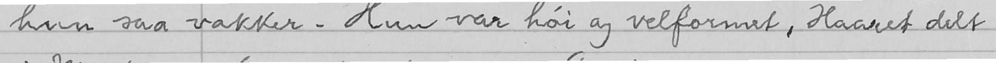hun saa vakker. Hun var høi og velformet, Haaret delt
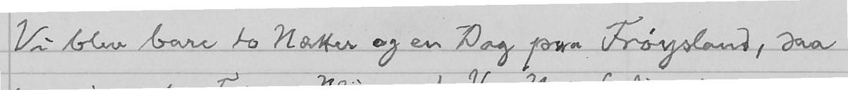Vi blev bare to Nætter og en Dag paa Frøysland, saa
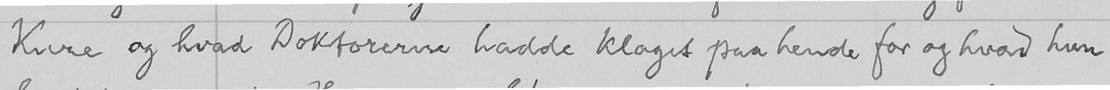Kure og hvad Doktorerne hadde klaget paa hende for og hvad hun
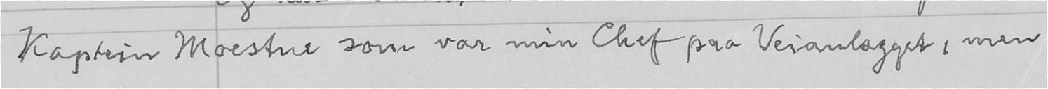Kaptein Moestue som var min Chef paa Veianlægget, men
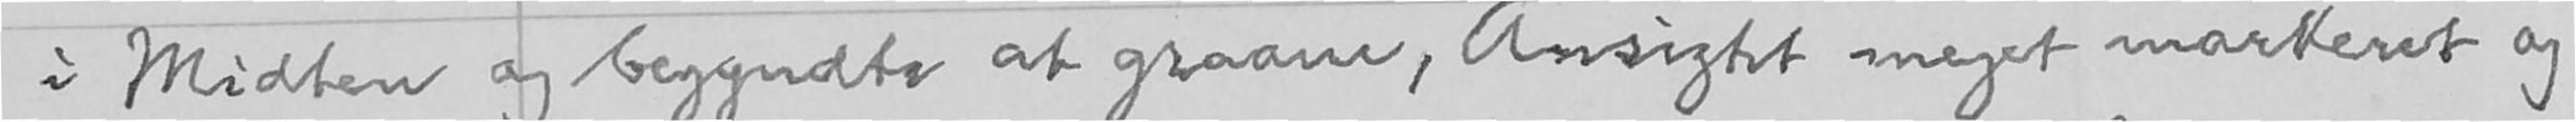i Midten og begyndte at graane, Ansigtet meget markeret og
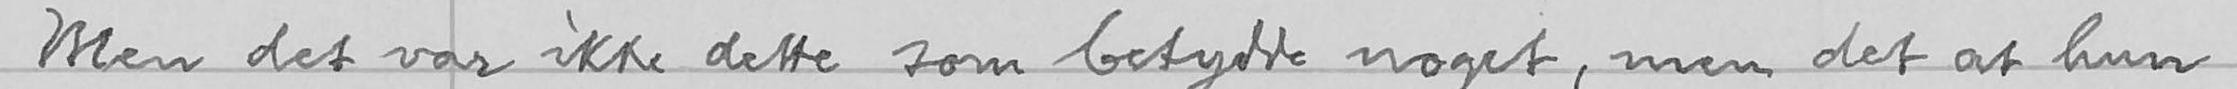Men det var ikke dette som betydde noget, men det at hun
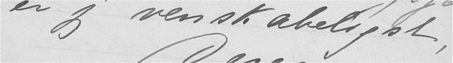er jeg venskabeligst,
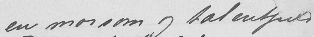en morsom og talentfuld
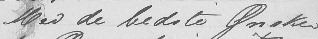Med de bedste Ønsker
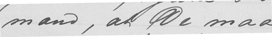mand, at De maa
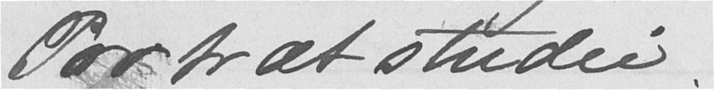Portrætstudie.
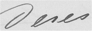Deres
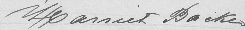Harriet Backer
